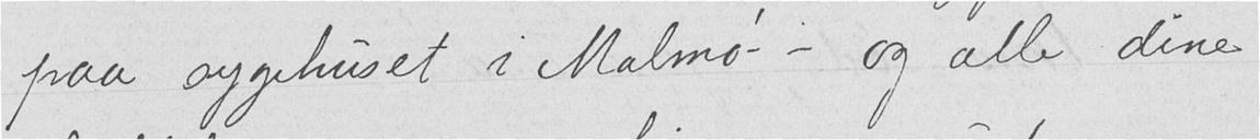paa sygehuset i Malmø - - og alle dine
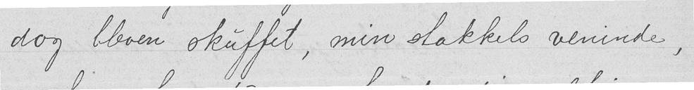dog bleven skuffet, min stakkels veninde,
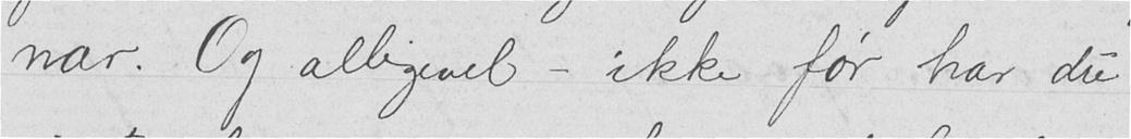nær. Og alligevel - ikke før har du
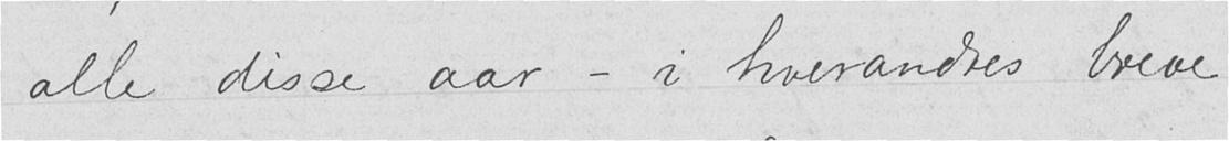alle disse aar – i hverandres breve
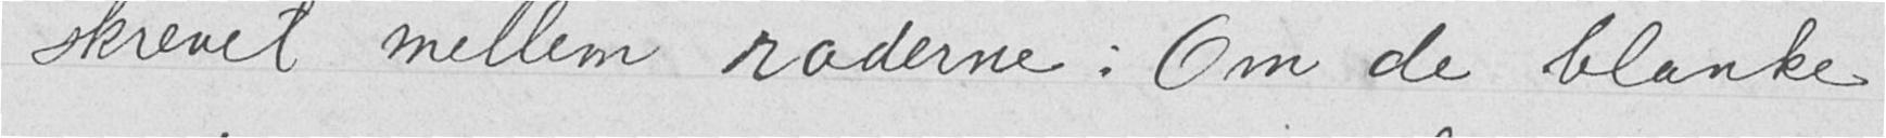skrevet mellem raderne: Om de blanke
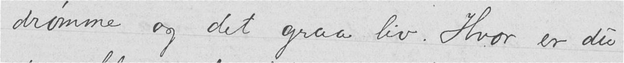drømme og det graa liv. Hvor er du
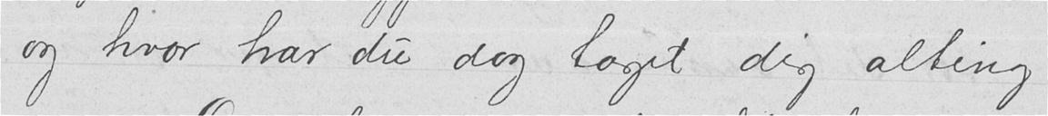og hvor har du dag taget dig alting
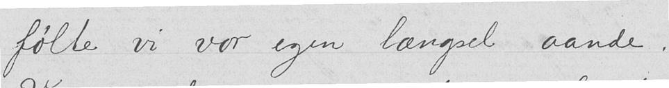følte vi vor egen længsel aande.
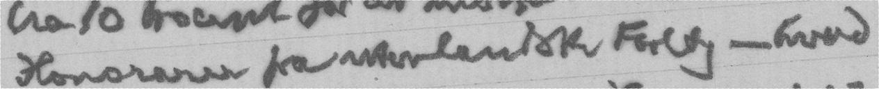Honorarer fra utenlandske Forlag - hvad
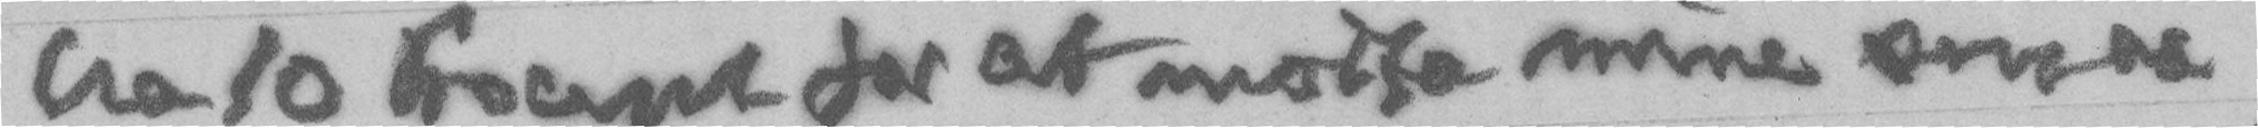ha 10 Procent for at motta mine smaa
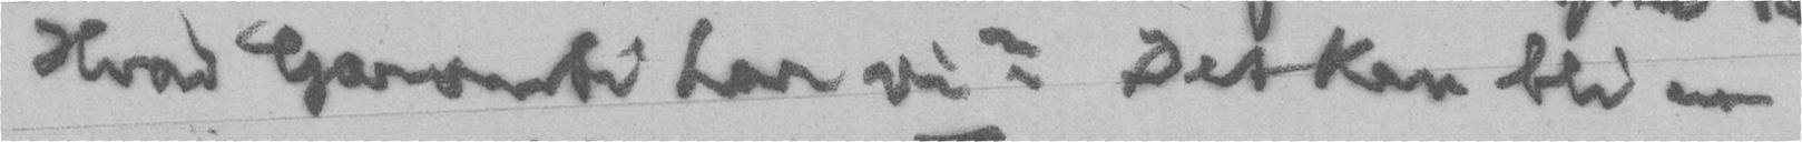Hvad Garanti har vi? Det kan bli en
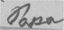Papa
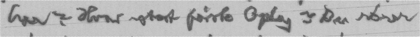har? Hvor stort første Oplag? Du rører
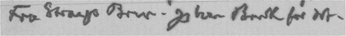Fru Strays Brev. Jeg har Bruk for det.
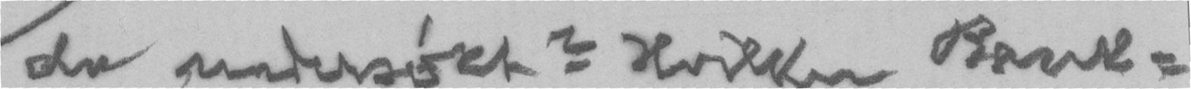du undersøkt? Hvilken Bank-
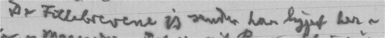De Fillebrevene jeg sender har ligget her i
